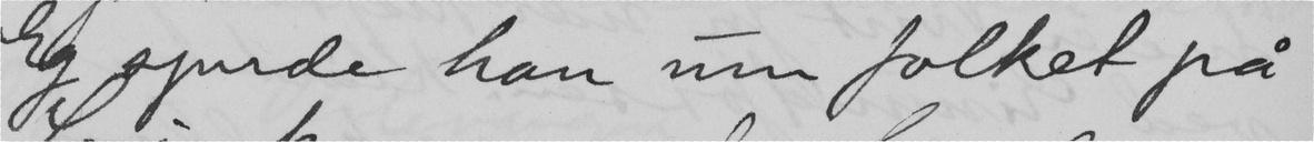Eg spurde han um folket på
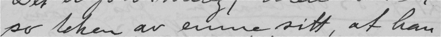so teken av emne sitt, at han
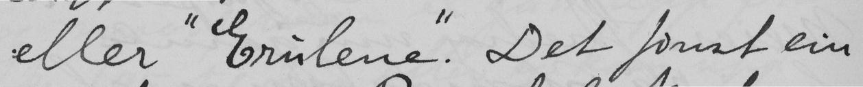eller "Erulene". Det finst ein
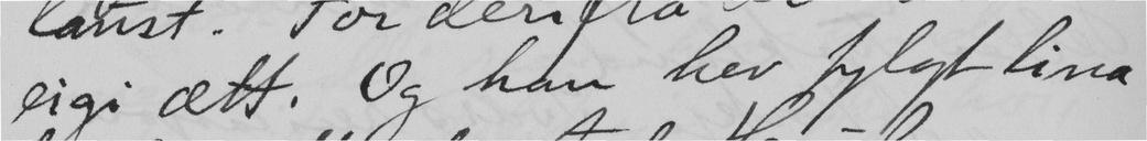eigi ætt. Og han hev fylgt lina
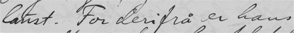laust. For deritrå er hans
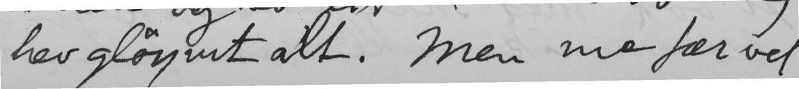hev glöymt alt. Men me fær vel
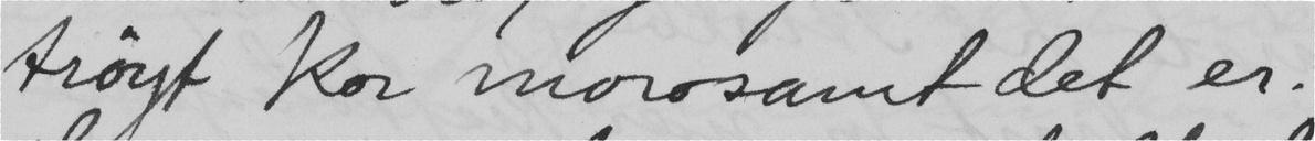tröygt kor morosamt det er.
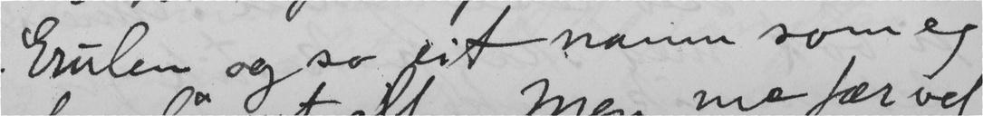Erulen og so sit namn som eg
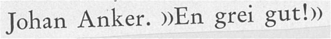Johan Anker. "En grei gut!"
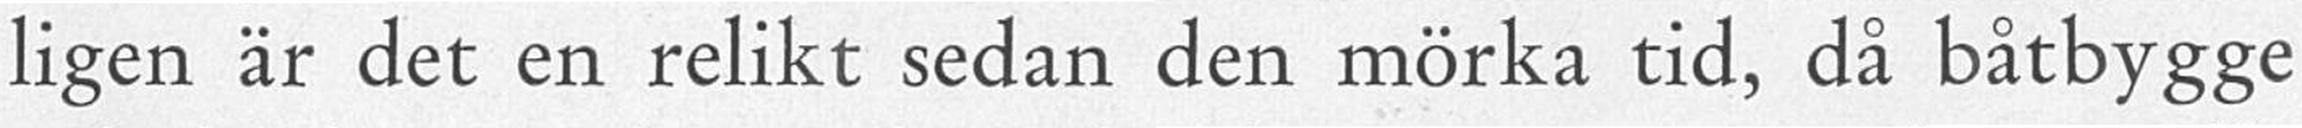ligen är det en relikt sedan den mörka tid, då båtbygge
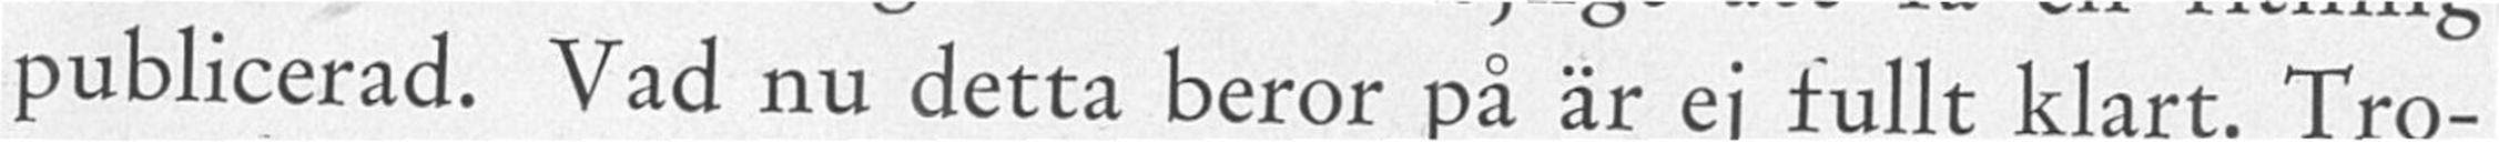publicerad. Vad nu detta beror på är ej fullt klart. Tro-
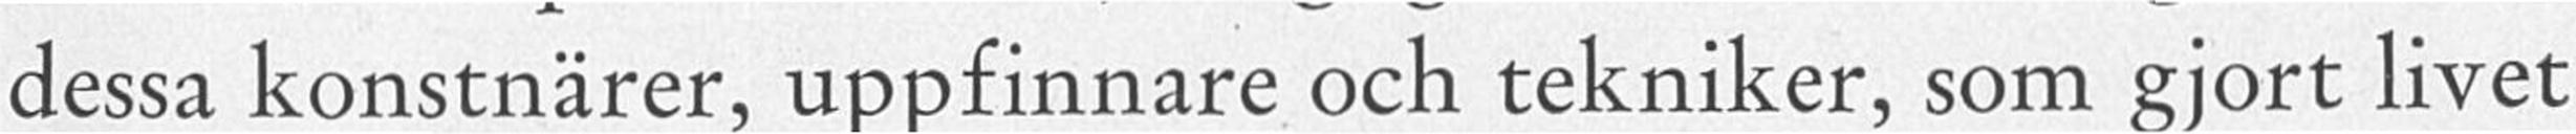dessa konstnärer, uppfinnare och tekniker, som gjort livet
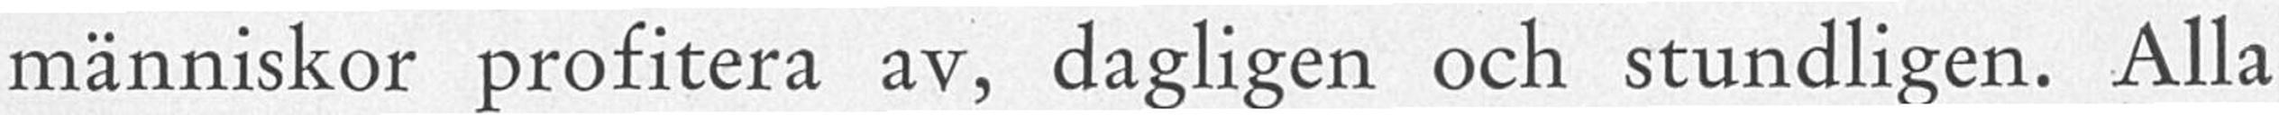människor profitera av, dagligen och stundligen. Alla
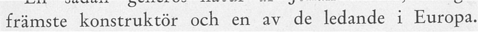främste konstruktör och en av de ledande i Europa.
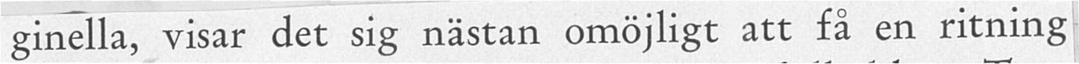ginella, visar det sig nästan omöjligt att få en ritning
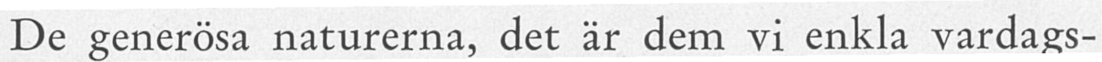De generösa naturerna, det är dem vi enkla vardags-
